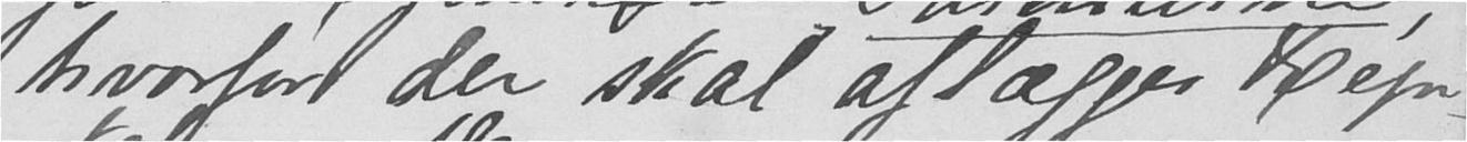hvorfor der skal aflægges Regn-
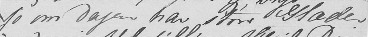jo om dagen har store Glæder
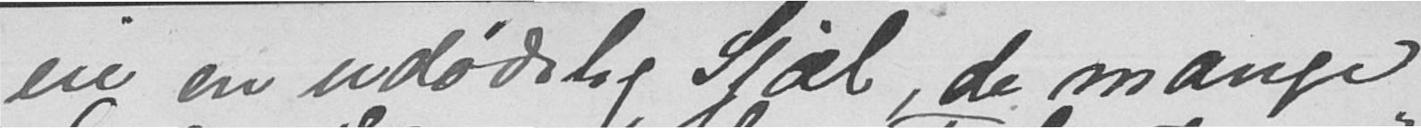eie en udødelig Sjæl, de mange
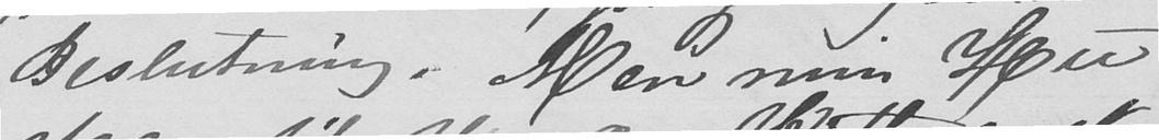Beslutning. Men min Hu
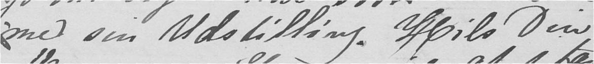med sin Udstilling. Hils Din
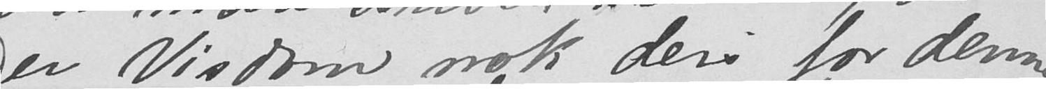er Visdom nok deri for denne
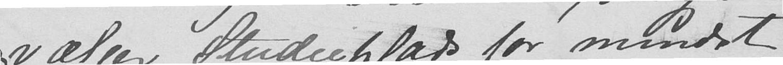vælger Studieplads for mindst
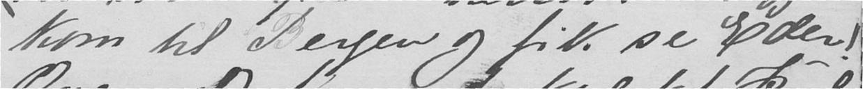kom til Bergen og fik se Eder!
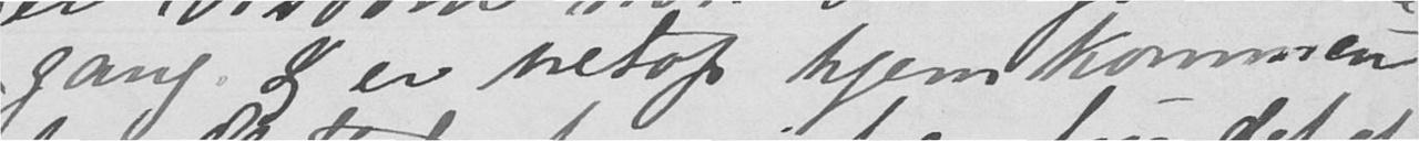gang. Jeg er netop hjemkommen
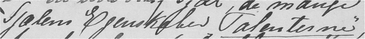Sjælens Egenskaber "Talenterne",
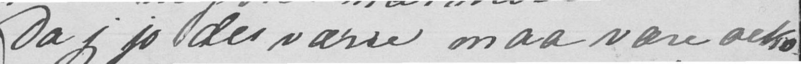Da jeg jo desværre maa være oeko-
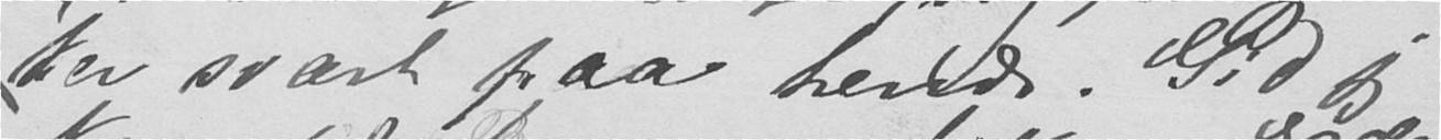ker svært paa hende. Gid jeg
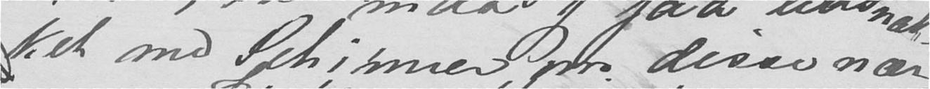ket med Schirmer om disse nær-
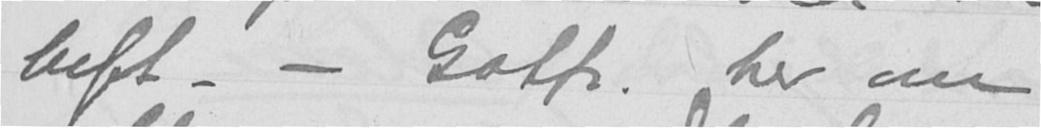luft. - Gotfr. her om
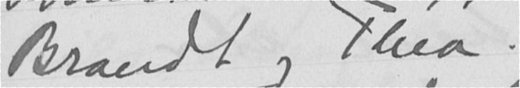Brandt og Thea.
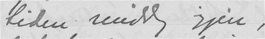Liden middag igjen,
